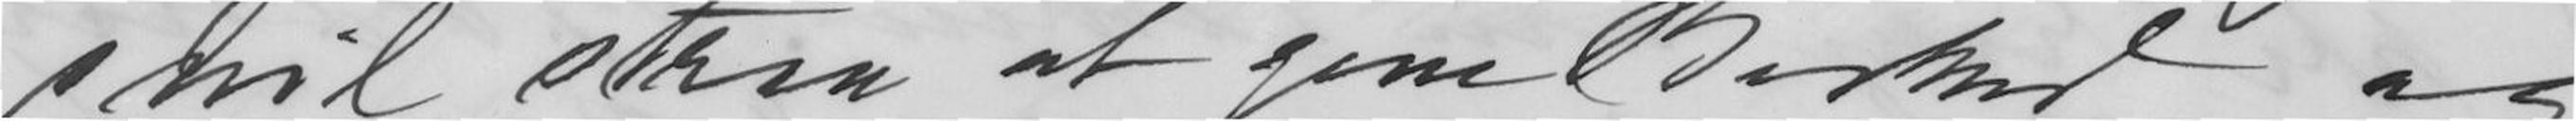snil strax at give Besked og
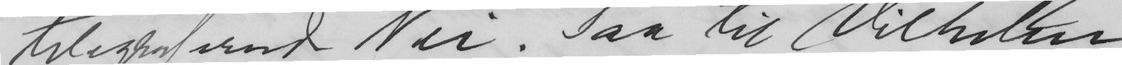telegraferede Nei! Saa til Vilhelm
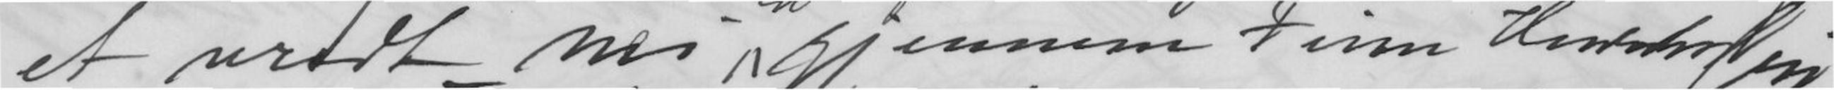et uredt nei til mig gjennem Finn Henrikssen
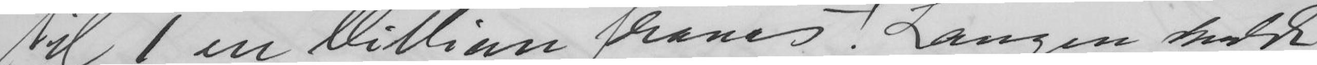til 1 en million francs! Langen skulde
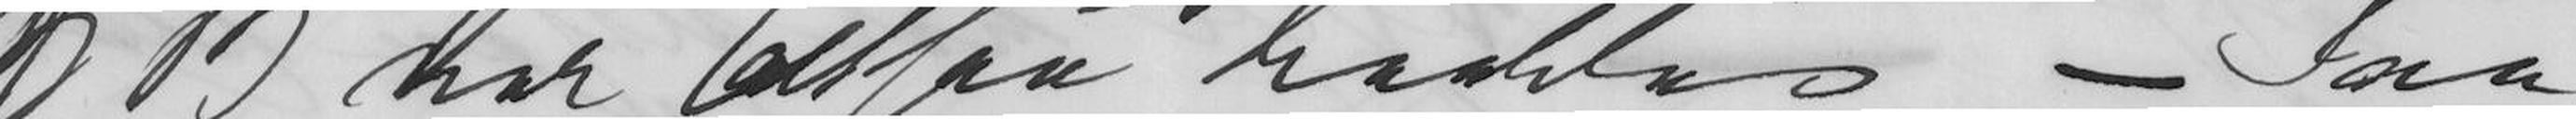B B var altsaa haabløs. - Saa
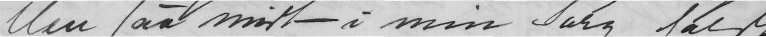Men saa midt i min Sorg faldt
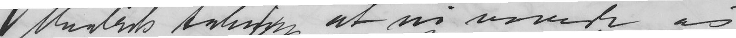theatrets Aabning at vi vovede os
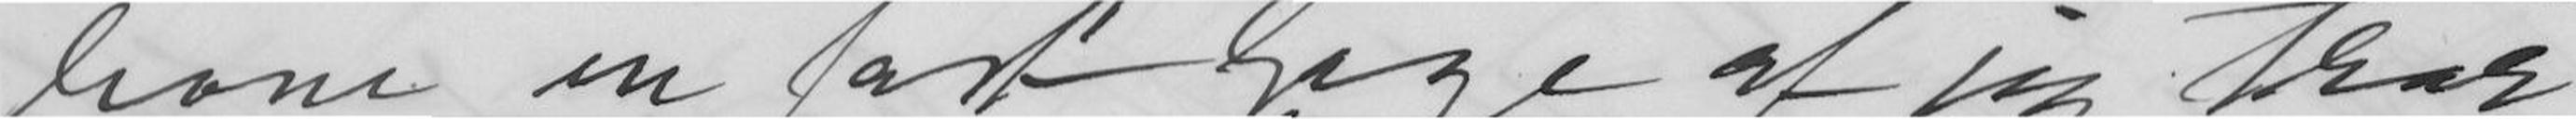have en fast Gage af jeg tror
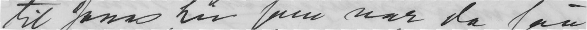til Jonas Lie som var da saa
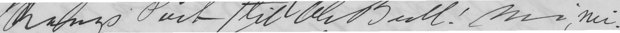Rangs Pust til Ole Bull! Nei, Nei.
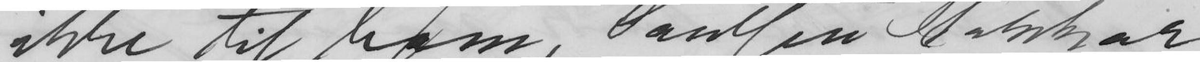ikke til ham, Paulsen Stakkar
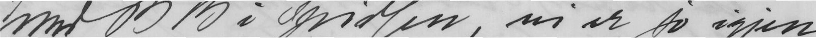med B B i Spidsen, vi er jo igjen
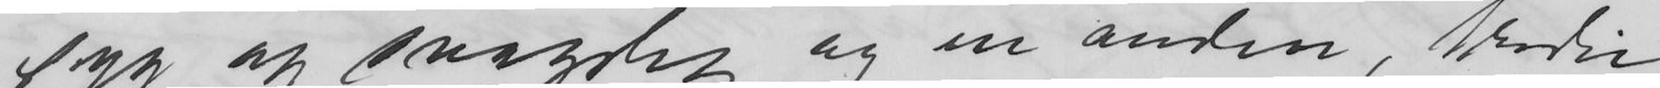syg og svagelig og en anden, tredie
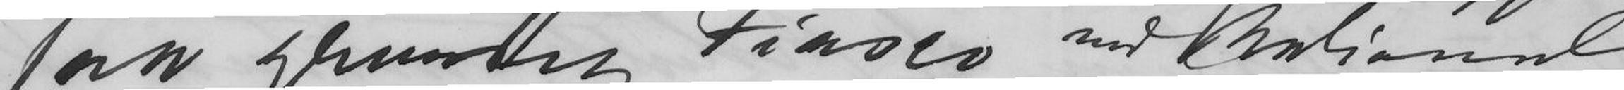saa grundig Fiasco ved National-
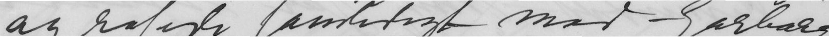og rasede samtidigt mod Garborg
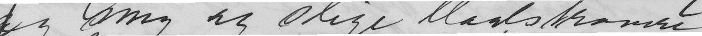og mig og slige Maalstrævere!
